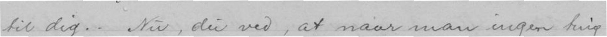til dig. Nu, du ved, at naar man ingen ting
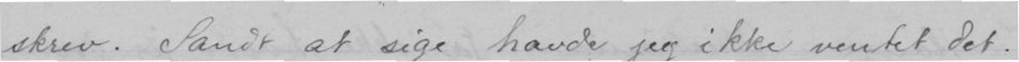skrev. Sandt at sige havde jeg ikke ventet det.
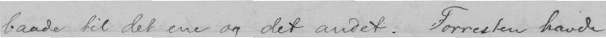baade til det ene og det andet. Forresten havde
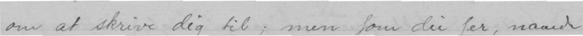om at skrive dig til, men som du ser, naaede
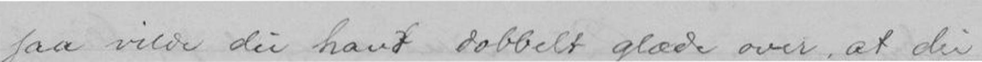saa vilde du havt dobbelt glæde over at du
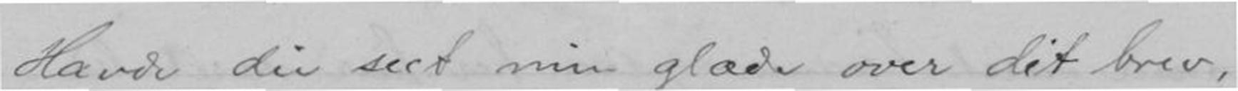Havde du seet min glæde over dit brev,
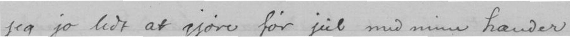jeg jo lidt at gjøre før jul med mine hænder
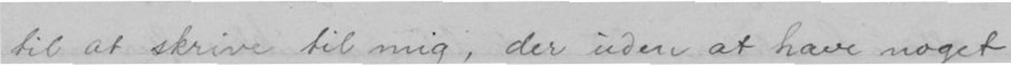at skrive til mig, der uden at have noget
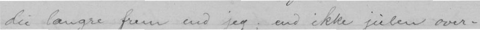du længre frem end jeg, end ikke julen over-
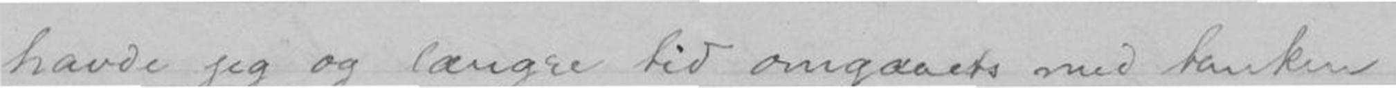havde jeg og længre tid omgaaets med tanken
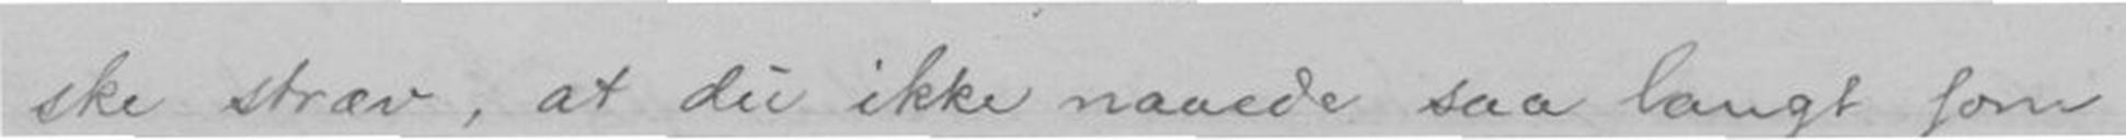stræv, at du ikke naaede saa langt som
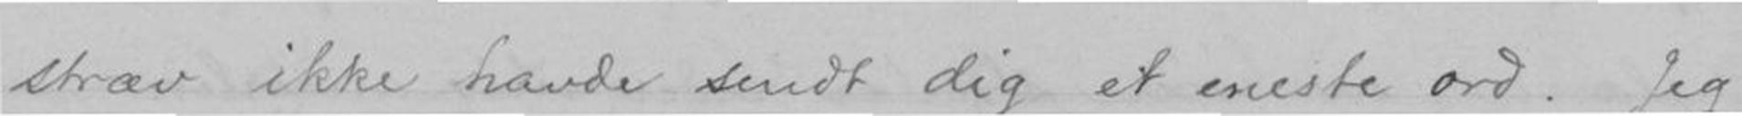stræv ikke havde sendt dig et eneste ord. Jeg
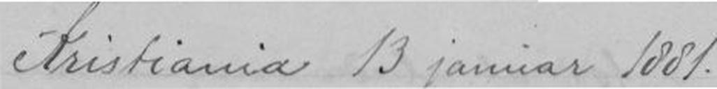Kristiania 13 januar 1881.
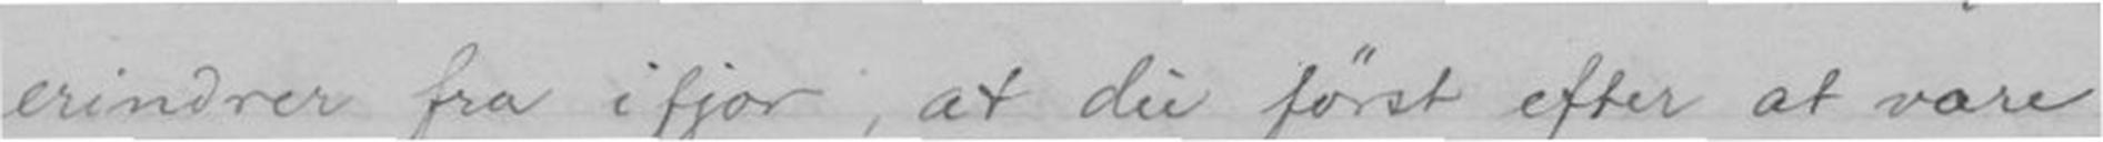erindrer fra ifjor, at du først efter at være
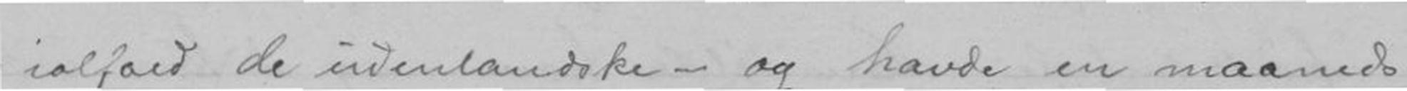- ialfald de udenlandske - og havde en maaneds
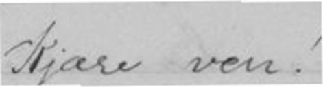Kjære ven!
